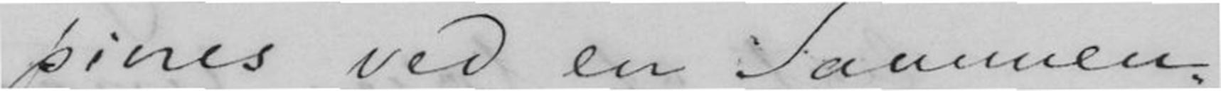pines ved en Sammen-
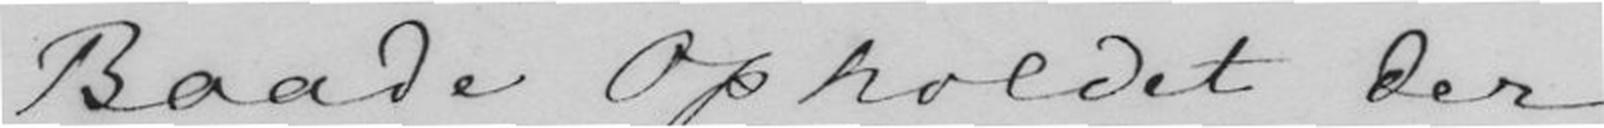Baade Opholdet der
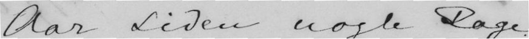Aar siden nogle Dage.
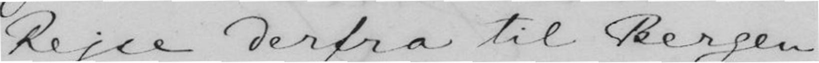Rejse derfra til Bergen
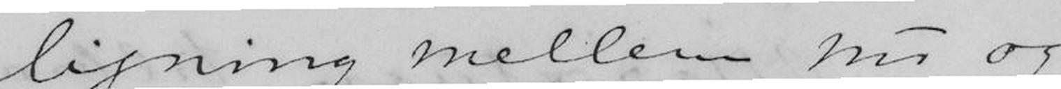ligning mellem nu og
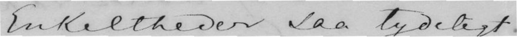Enkeltheder saa tydeligt
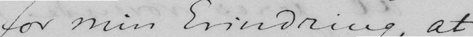for min Erindring, at
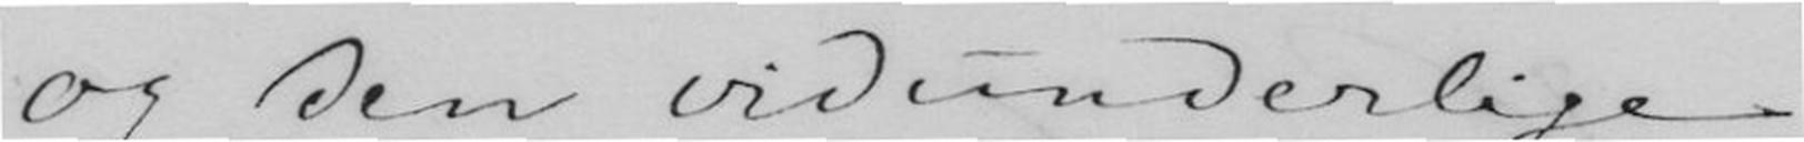og den vidunderlige
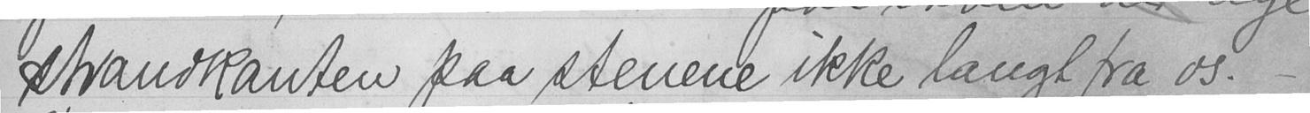strandkanten paa stenene ikke langt fra os. -
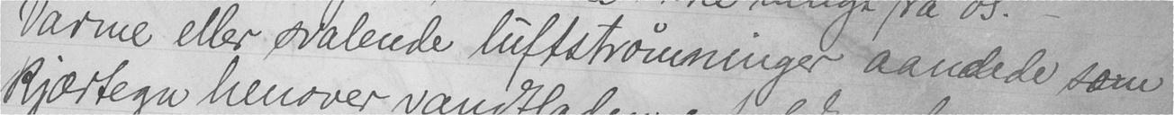Varme eller svalende luftstrømninger aandede som
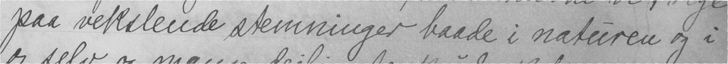paa vekslende stemninger baade i naturen og i
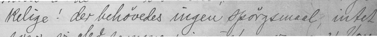kelige! der behøvedes ingen spørgsmaal, intet
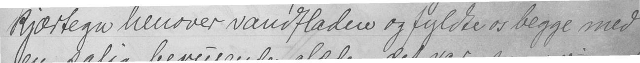kjærtegn henover vandfladen og fyldte os begge med
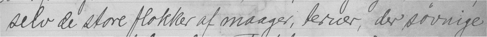selv de store flokker af maager, terner, der søvnige
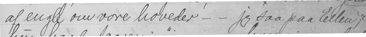af engle om vore hoveder - - jeg saa paa Ellen;
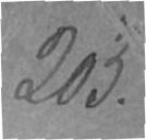203.
